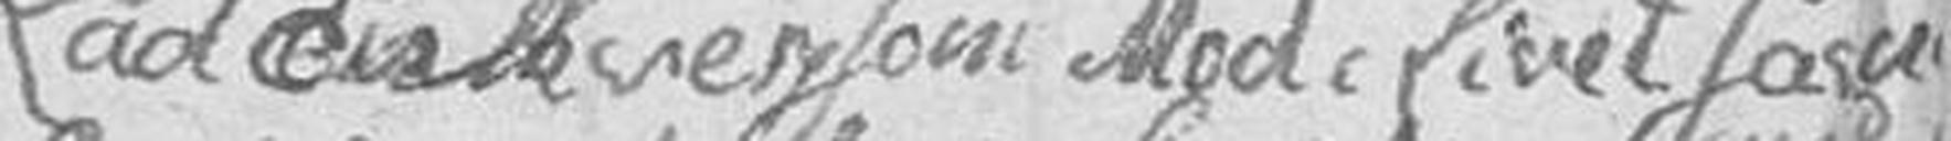Lad  Mod i Livet savn
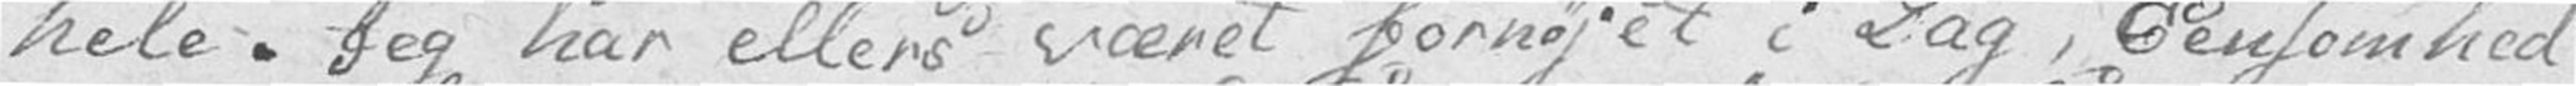hele. Jeg har ellers været fornøjet i Dag, Eensomhed
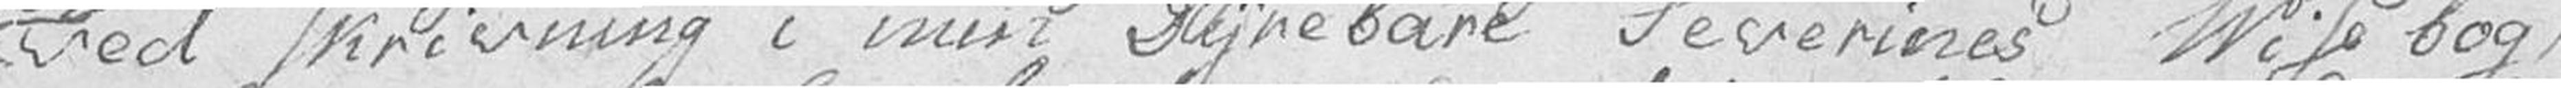ved skrivning i min Dyrebare Severines Vise bog,
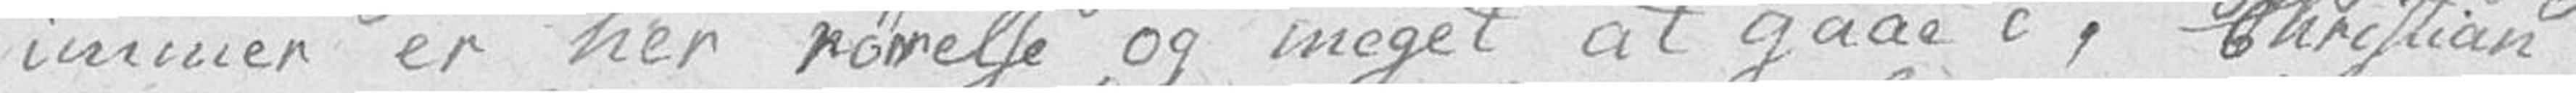immer er her rørelse og meget at gaae i, Christian
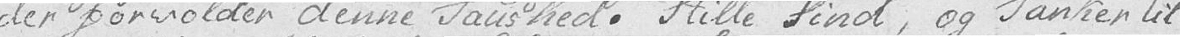der forvolder denne Taushed. Stille Sind, og Tanker til
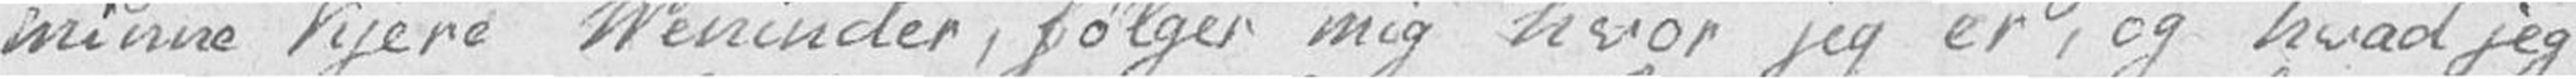minne kjere Veninder, følger mig hvor jeg er, og hvad jeg
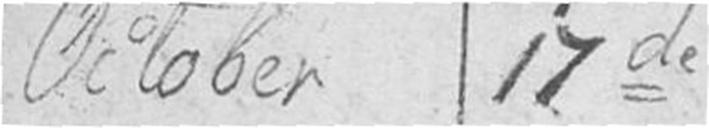October 17de
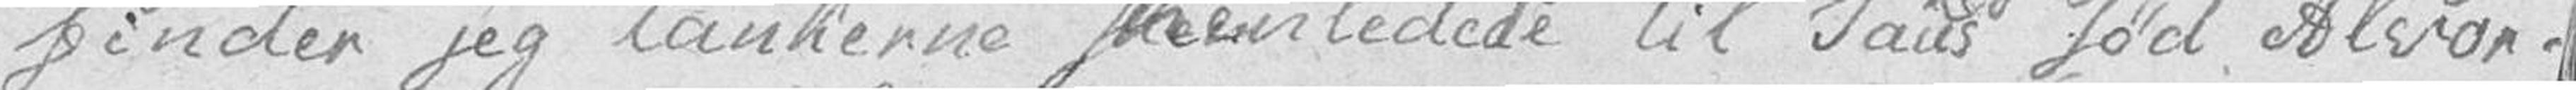finder jeg tankerne henledede til Taus sød Alvor.
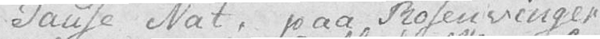Tause Nat! paa Rosenvinger
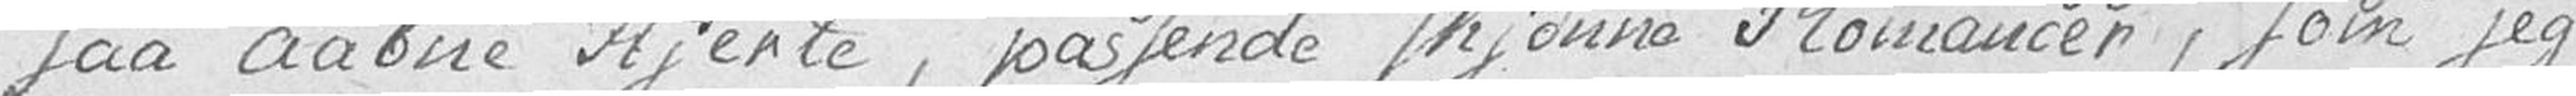saa aabne Hjerte, passende skjønne Romancer, som jeg
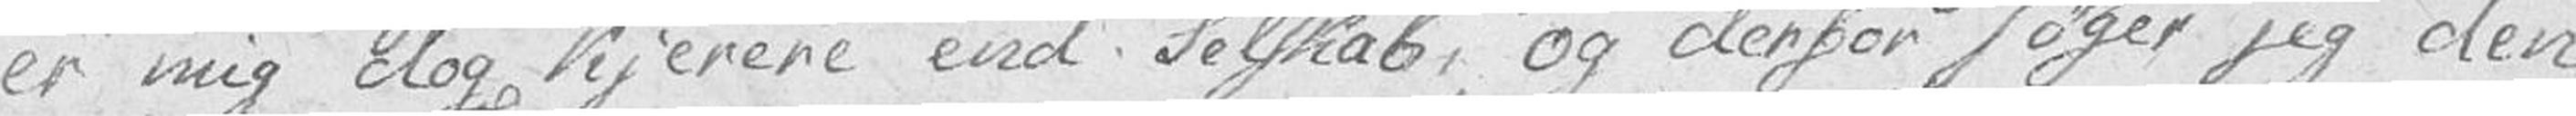er mig dog kjerere end Selskab, og derfor søger jeg den
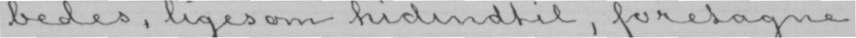bedes, ligesom hidindtil, foretagne
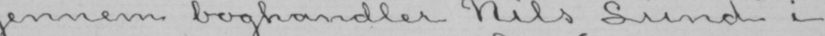gennem boghandler Nils Lund i
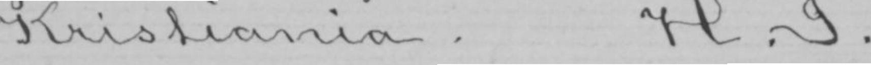Kristiania. H. I.
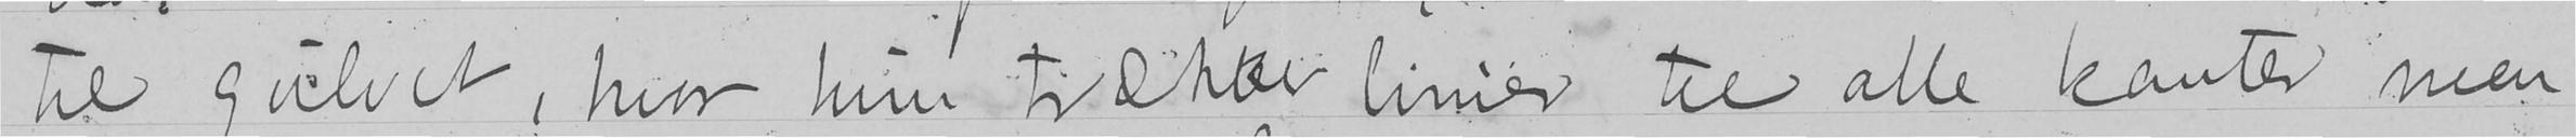til gulvet, hvor hun trækker linier til alle kanter men
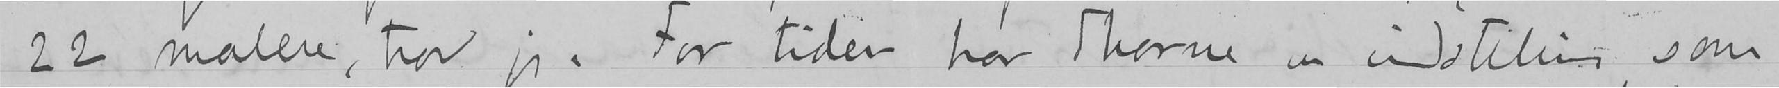22 malere, tror jeg. For tiden har Thorne en udstilling som
